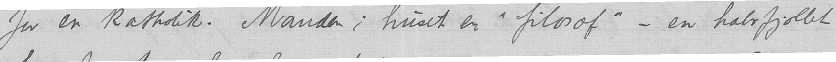for en katholik. Manden i huset en "filosof" - en halvfjollet
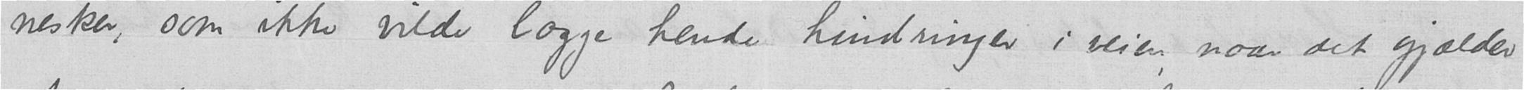nesker, som ikke vilde lægge hende hindringer i veien, naar det gjælder
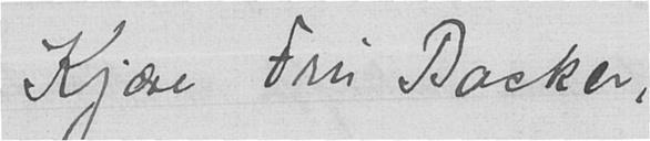Kjære Fru Backer,
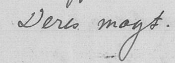Deres magt.
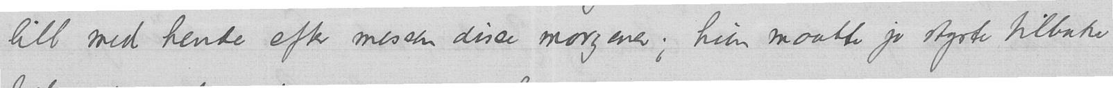litt med hende efter messen disse morgener; hun maatte jo styrte tilbake
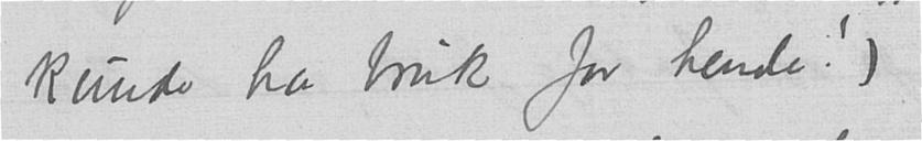kunde ha bruk for hende!)
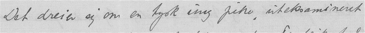Det dreier sig om en tysk ung pike, uteksamineret
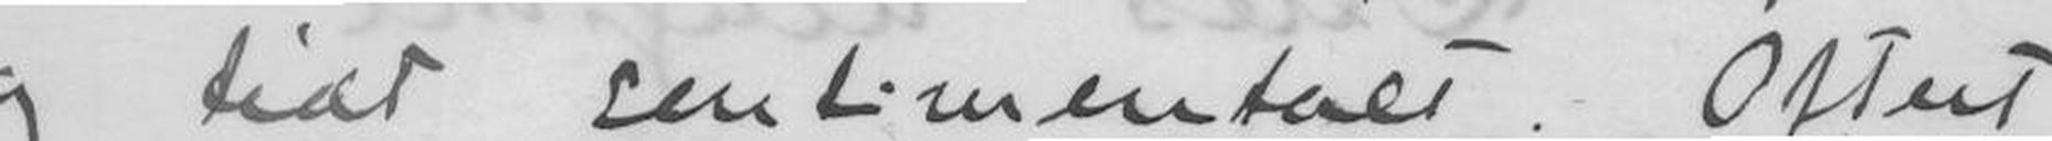og tidt sentimentalt. Oftest
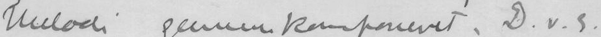Melodi gennemkomponerer. D.v.s.
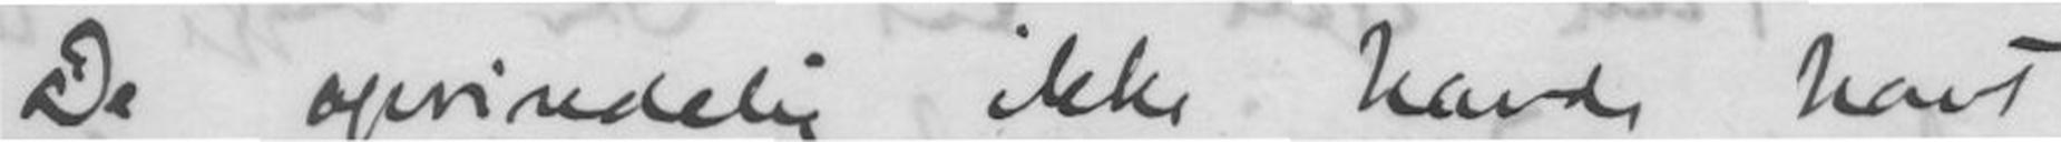De oprindelig ikke havde havt
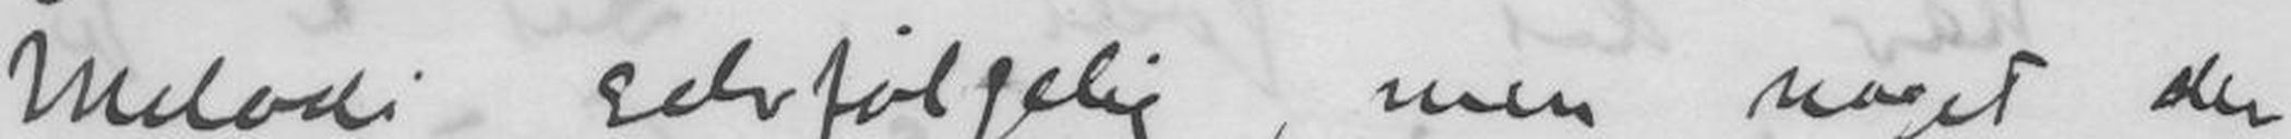Melodi selvfølgelig, men noget der
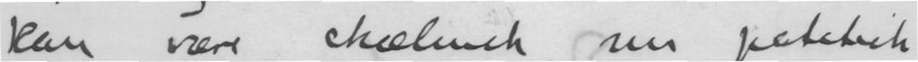kan være chælmsk, ren patetisk
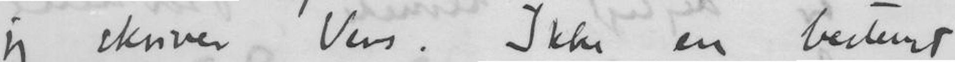jeg skriver Vers. Ikke en bestemt
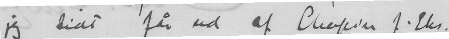jeg tidt får ud af Chopin f. Eks.)
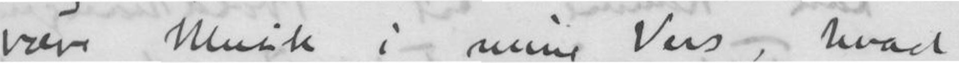være Musik i mine Vers, hvad
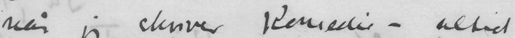- når jeg skriver Komedie - altid
